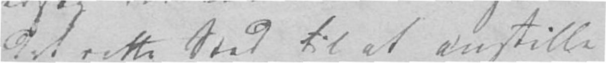det rette Sted til at anstille
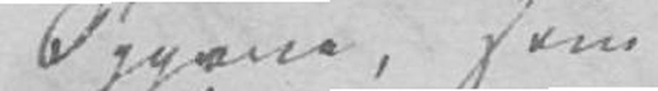Opgave, som
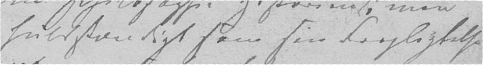fuldstændigt som sin Forpligtelse
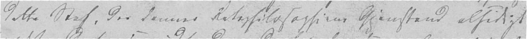dette Stof, der danner Retsphilosophiens Gjenstand alsidigt
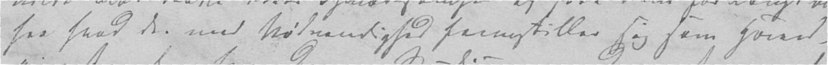fra hvad der med Nødvendighed fremstiller sig som Hoved-
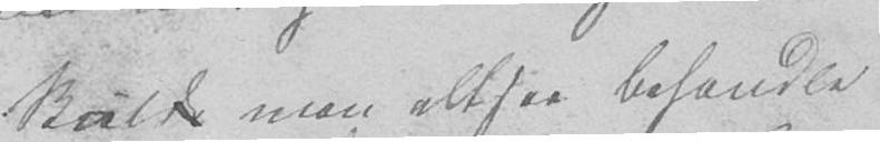Skal man altsaa behandle
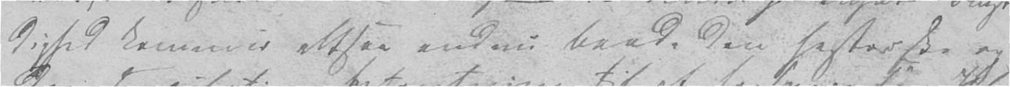dighed kommer altsaa endnu baade den historiske og
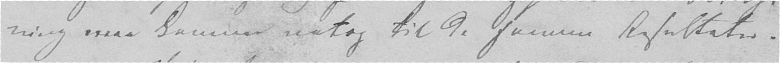ning man kommer netop til de samme Resultater.
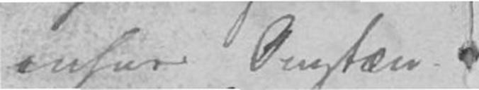enhver Omstæn-
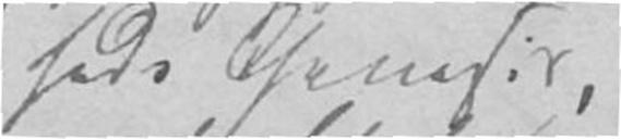heds Genesis,
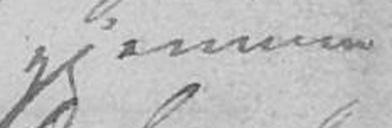gjennem
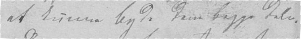at kunne byde Dem begge Dele.
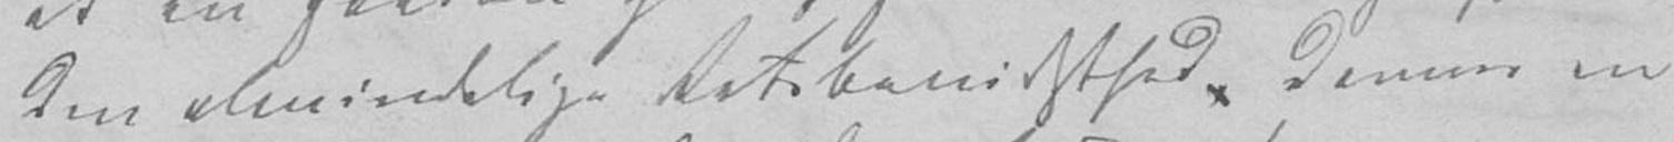den almindelige Retsbevidsthed danner en
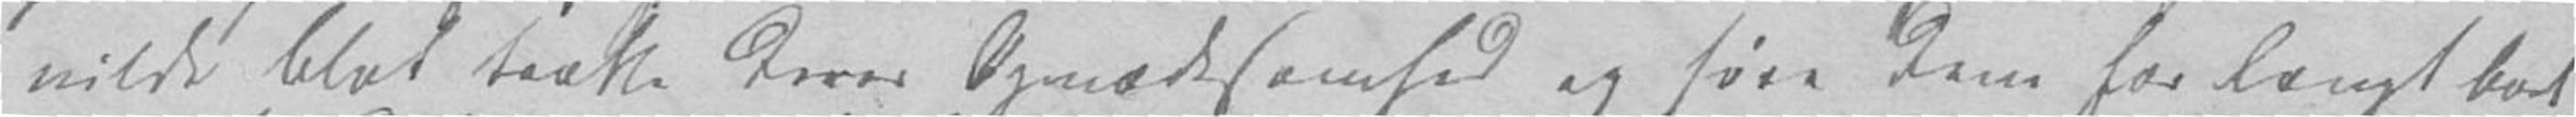vilde blot trætte Deres Opmærksomhed og føre Dem for langt bort
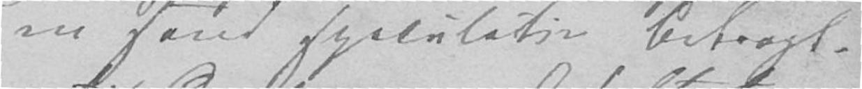en sand speculativ Betragt-
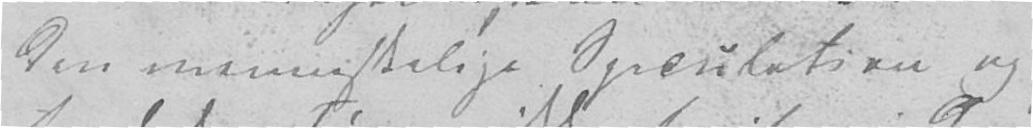den menneskelige Speculation og
